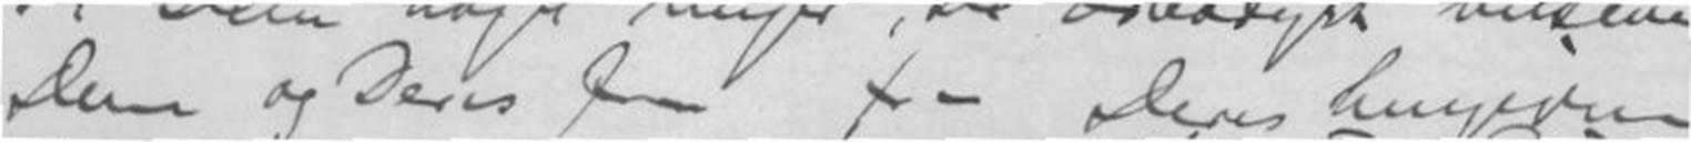Dem og Deres frue fra Deres hengivne
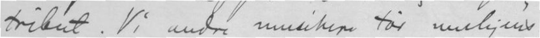tribut. Vi andre musikere tør muligens
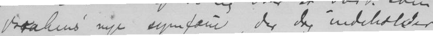Brahms nye symfoni, der dog indeholder
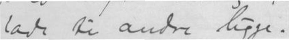lade ti andre ligge.
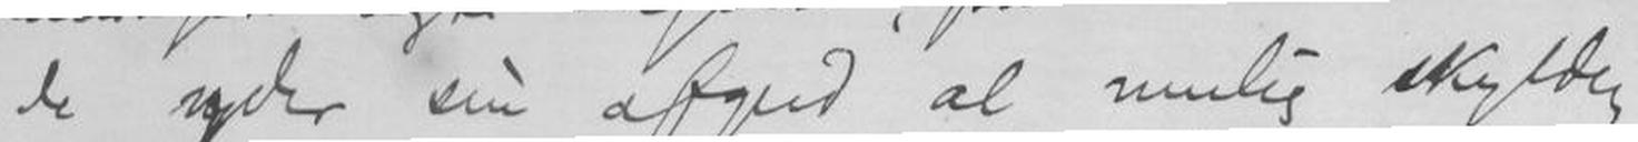de yder sin afgud al mulig skyldig
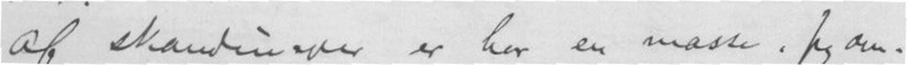Af skandinaver er her en masse. Jeg om-
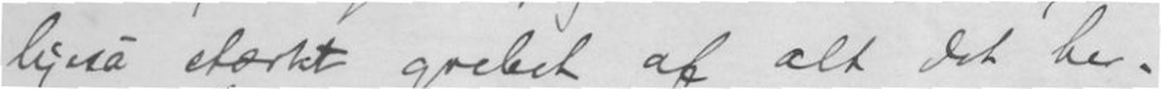ligeså stærkt grebet af alt det her-
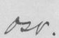osv.
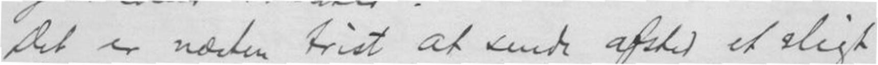Det er næsten trist at sende afsted et sligt
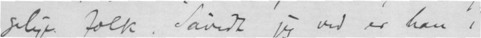gelige folk. Såvidt jeg ved er han i
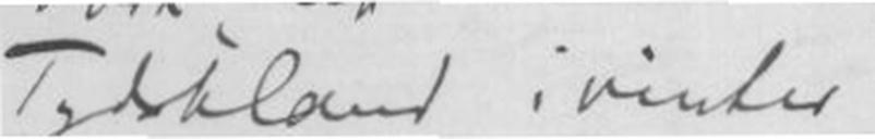Tydskland i vinter
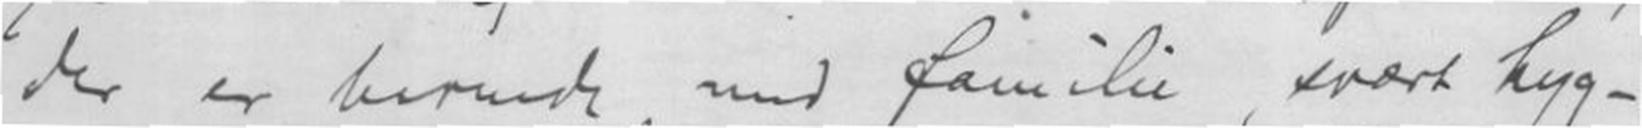der er hernede med familie, svært hyg-
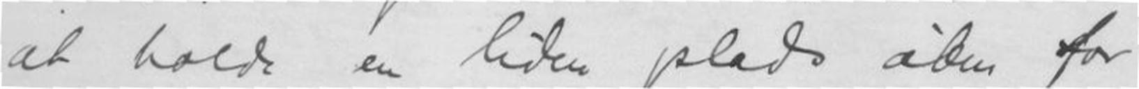at holde en liden plads åben for
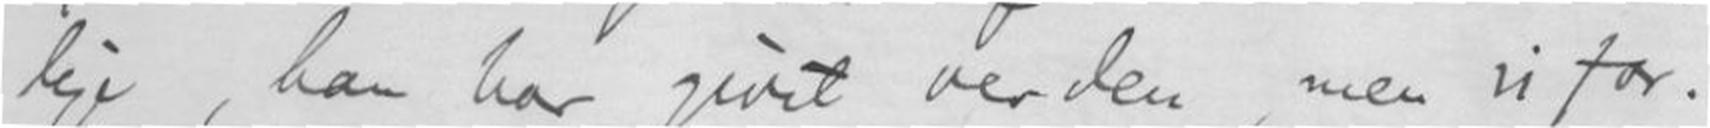lige, han har givet verden, men vi for-
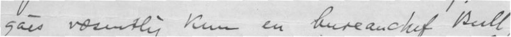gås væsentlig kun en bureauchef Bull,
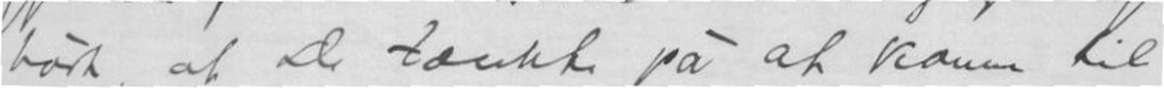hørt at De tænkte på at komme til
